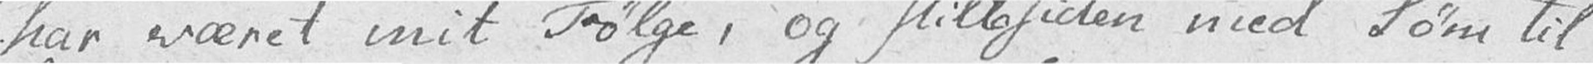har været mit Følge, og stillesiden med Søm til
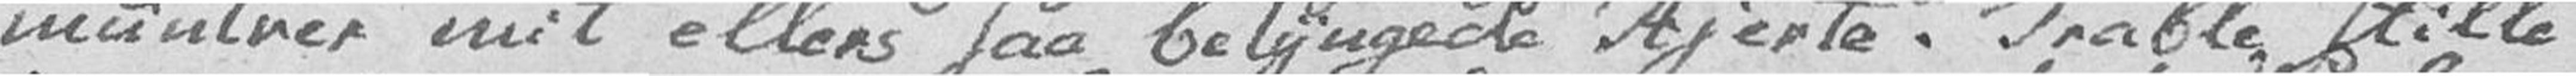muntrer mit ellers saa betyngede Hjerte. Trable, stille
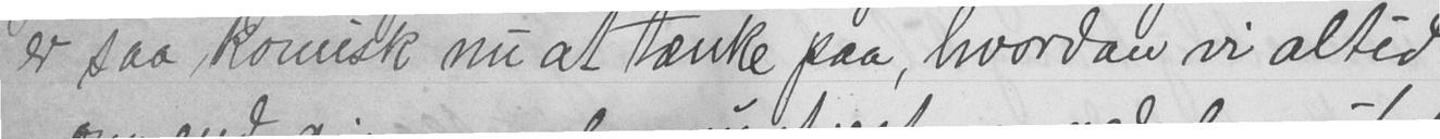er saa komisk nu at tænke paa, hvordan vi altid
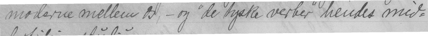moderne mellem os, - og "de tyske verber" hendes mid-
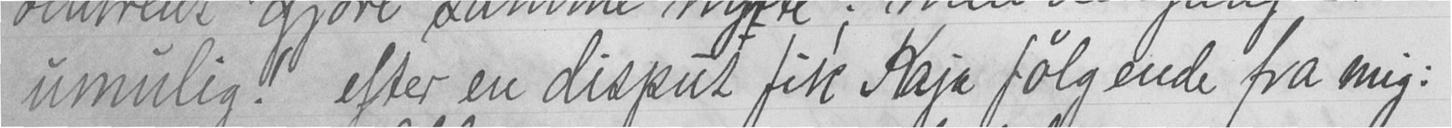umulig! efter en disput fik Kaja følgende fra mig:
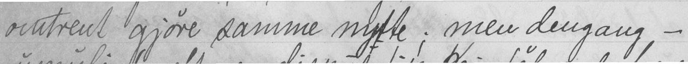omtrent gjøre samme nytte; men dengang -
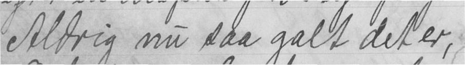Aldrig nu saa galt det er,
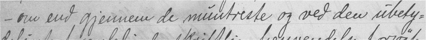- om end, gjennem de muntreste og ved den ubety-
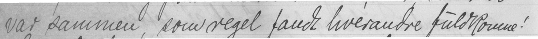var sammen, som regel fandt hverandre fuldkomne!
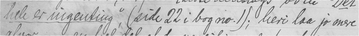hele er ingenting", (side 22 i bog no. 1); heri laa jo mere
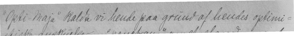"Opti-Maja" kaldte vi hende paa grund af hendes optimi-
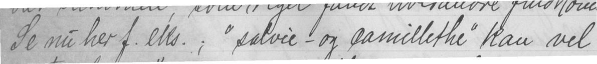Se nu her f. eks. ; "salvie- og camillethe" kan vel
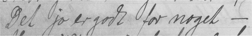Det jo er godt; for noget -
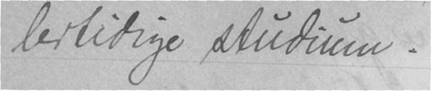lertidige studium.
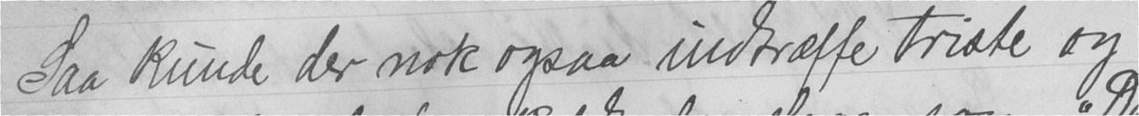Saa kunde der nok ogsaa indtræffe triste og
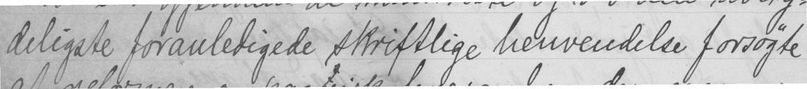deligste foranledigede skriftlige henvendelse forsøgte
